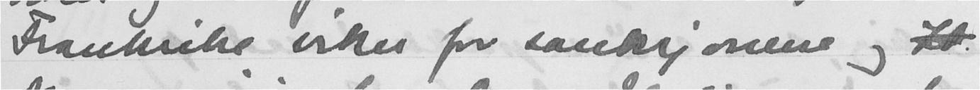Frankrike viker for sanksjonene og
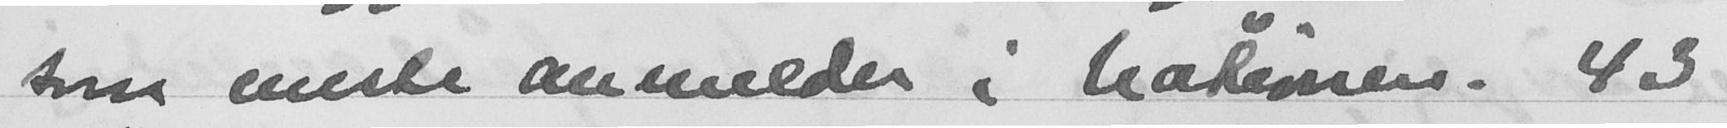som eneste anmelder i Nationen. 43
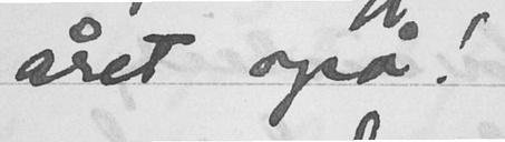året også!
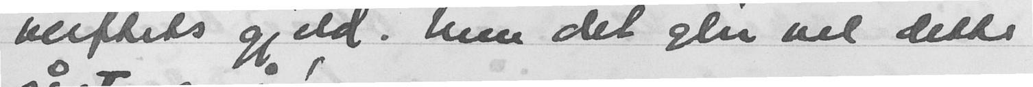verftets gjeld. Men det glir vel dette
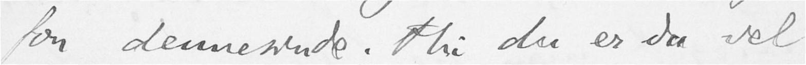for dennesinde; thi du er da vel
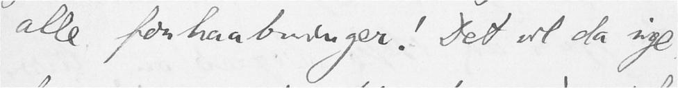alle forhaabninger! Det vil da sige
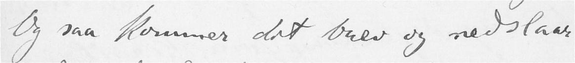Og saa kommer dit brev og nedslaar
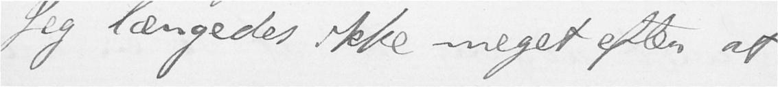Jeg længedes ikke meget efter at
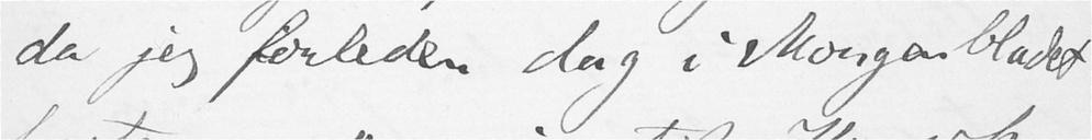da jeg forleden dag i Morgenbladet
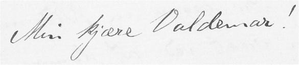Min kjære Valdemar!
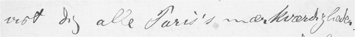vist dig alle Paris's mærkværdigheder.
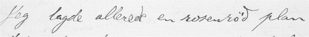Jeg lagde allerede en rosenrød plan
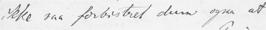ikke saa forbistret dum ogsaa at
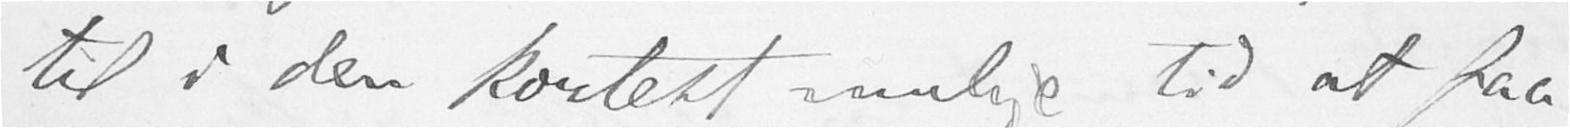til i den kortest mulige tid at faa
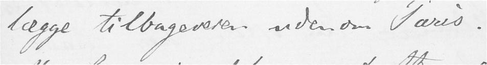lægge tilbageveien uden om Paris.
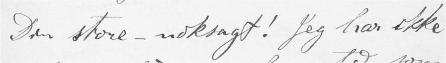Din store – noksagt! Jeg har ikke
